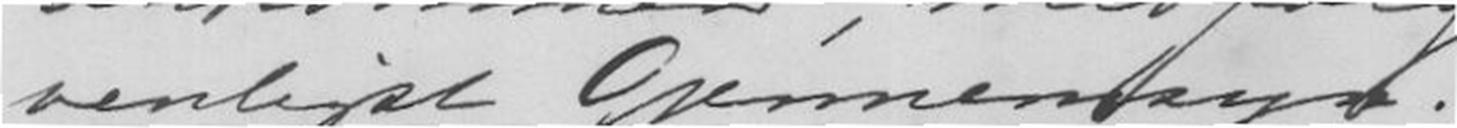venligst Gjennemsyn.
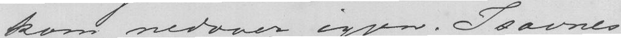kom nedover igjen. I savnes
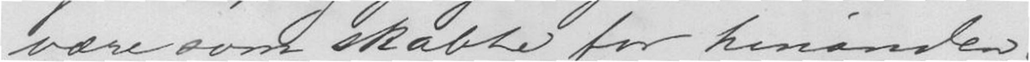være som skabte for hinanden,
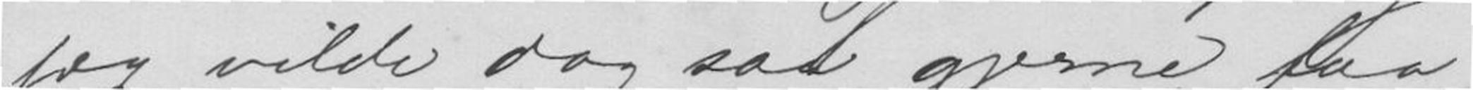jeg vilde dog saa gjerne faa
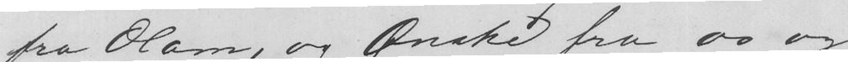fra Olaus, og Ønske fra os og
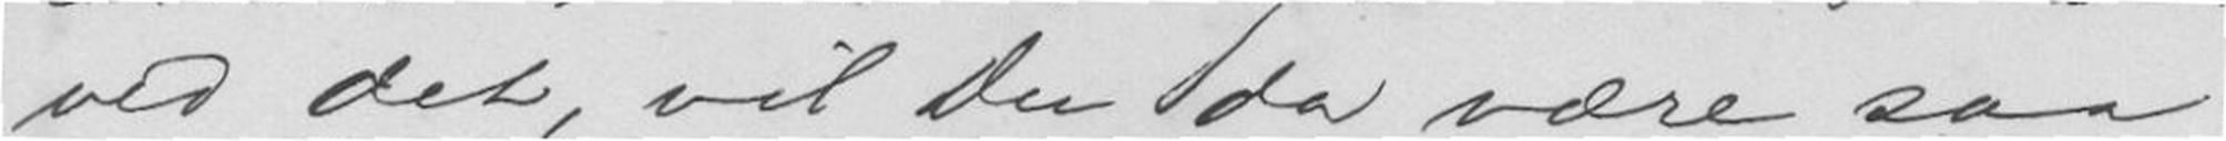ved det, vil du da være saa
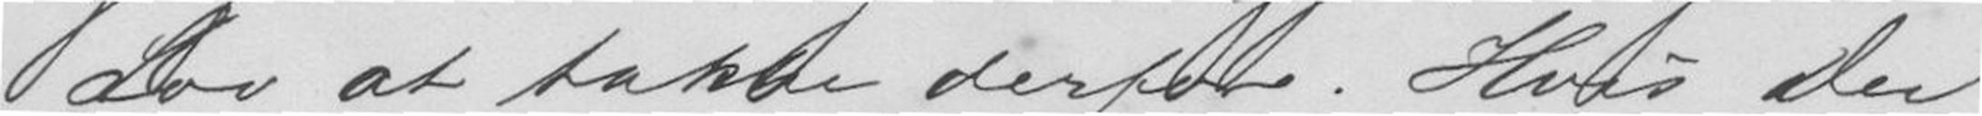Lov at takke derfor. Hvis du
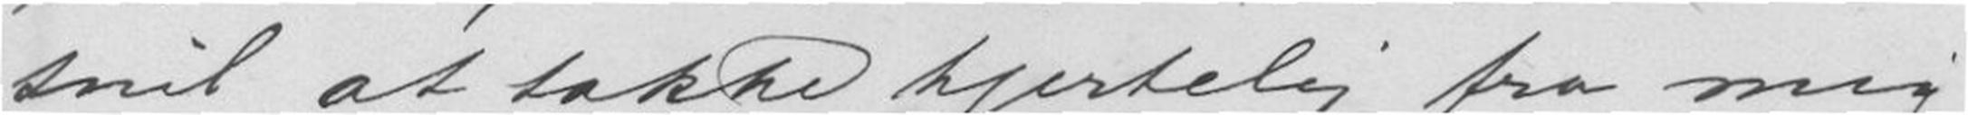snil at takke hjertelig fra mig,
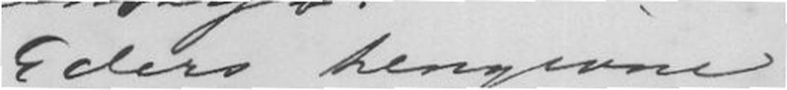Eders hengivne
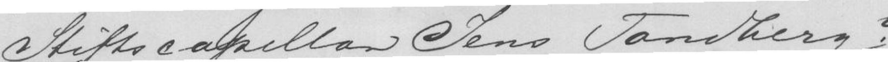Stiftskapellan Jens Tandberg?
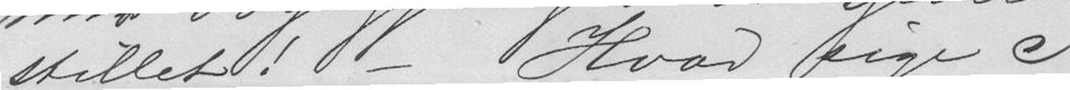stillet! --Hvad sige I
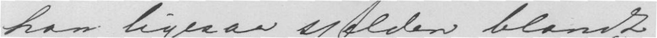han ligesaa sjelden blandt
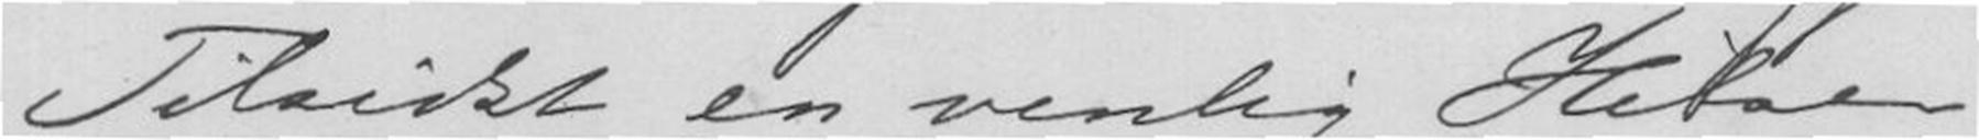Tilsidst en venlig Hilsen
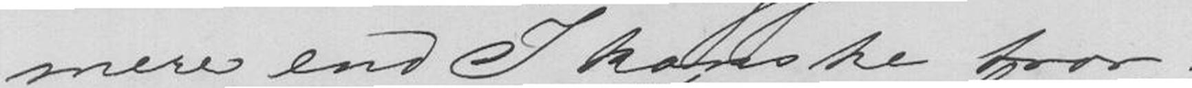mere end I kanske tror.
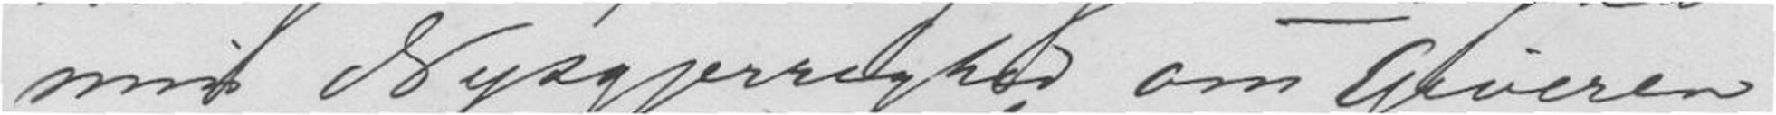min Nysgjerrighed om Giveren
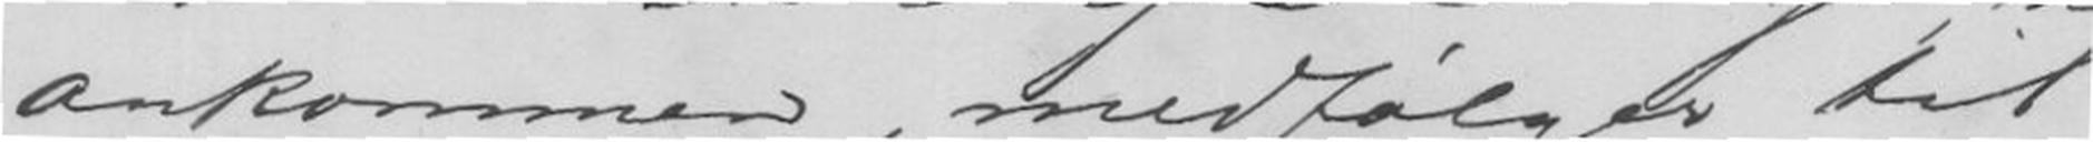ankommen, medfølger til
